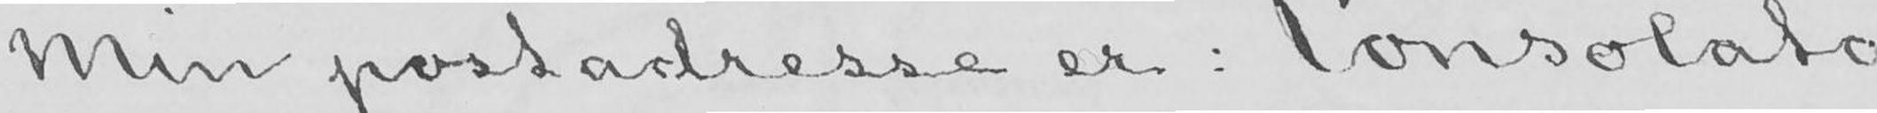Min postadresse er: Consolato
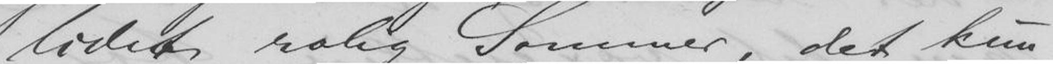lidet rolig Sommer, det kun-
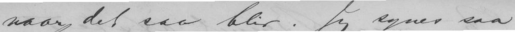naar det saa blir. Jeg synes saa
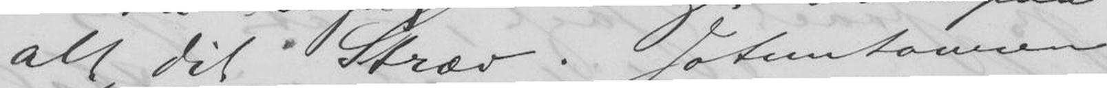alt Dit Stræv. Jotuntouren
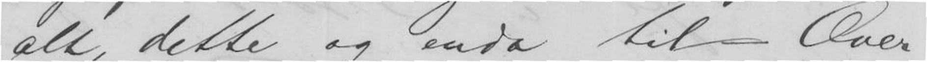alt dette og enda til - Over-
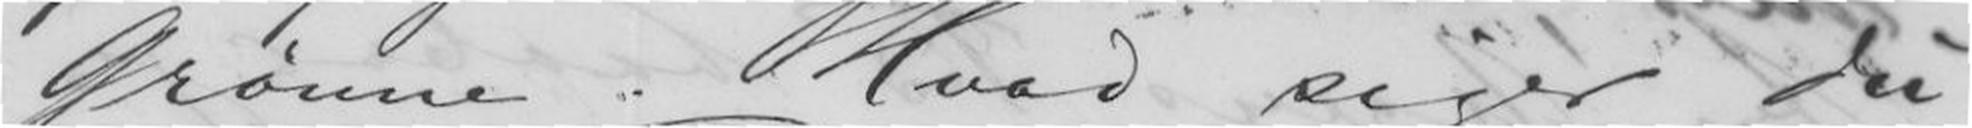Grønne. Hvad siger Du
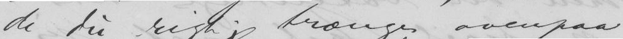de Du rigtig trænge ovenpaa
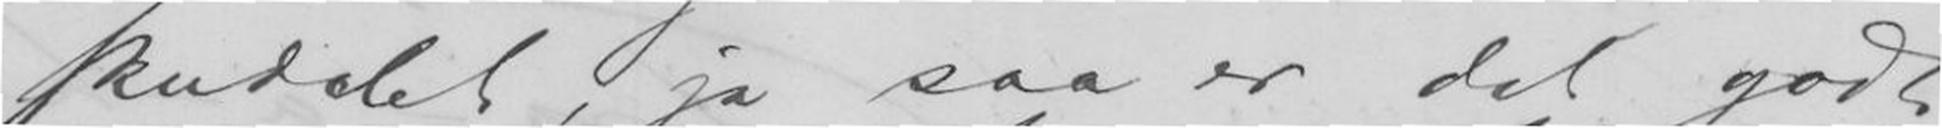skuddet, ja saa er det godt
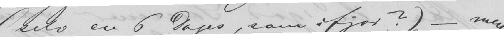(selv en 6 Dages, som ifjor?) - men
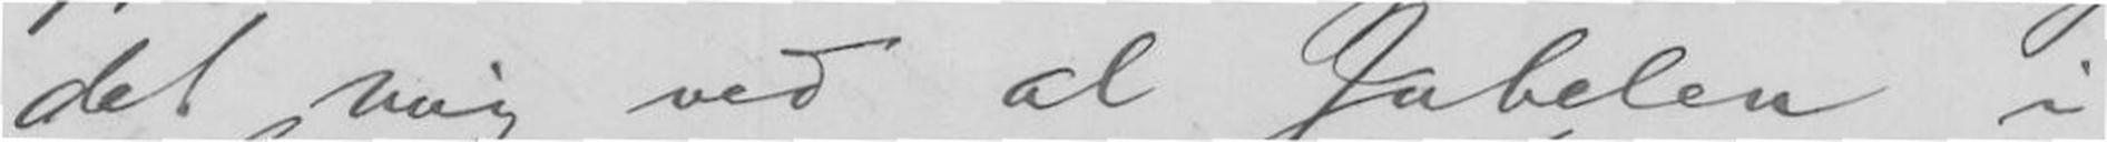det mig ved al Jubelen i
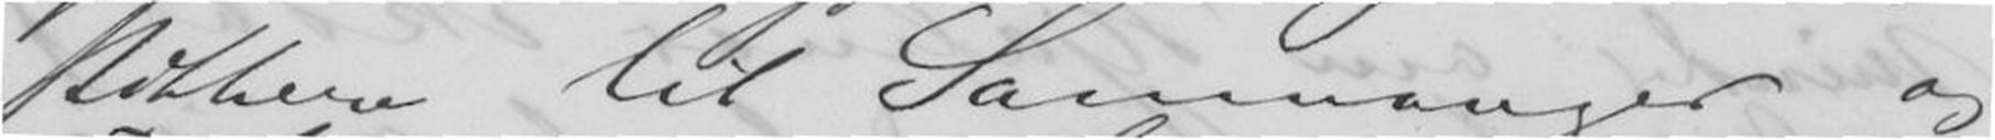stikkere til Samnanger og
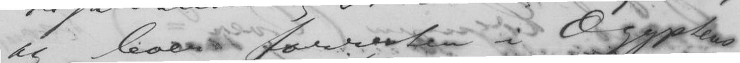og boer forresten i Ægyptens
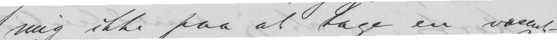mig ikke paa at tage en væsent-
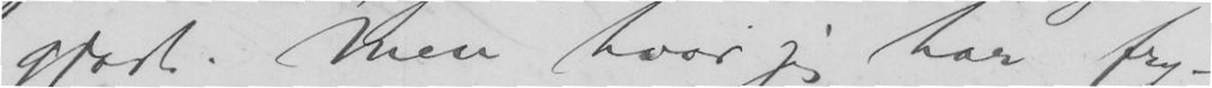gjort. Men hvor jeg har fry-
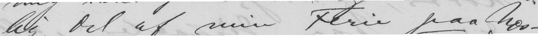lig Del af min Ferie paa Næs-
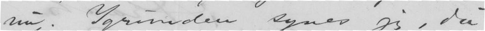nu. Igrunden synes jeg, Du
